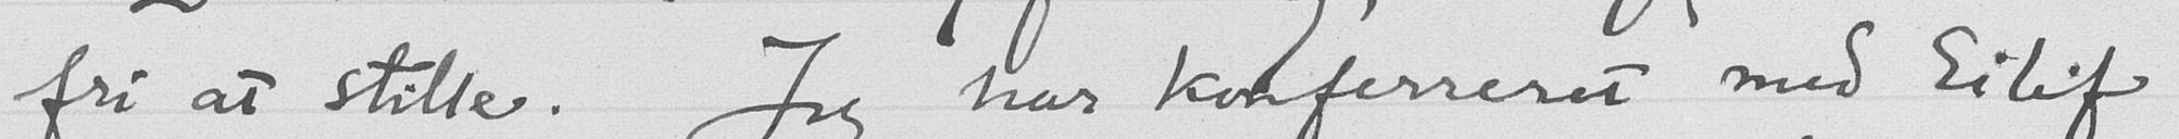fri at stille. Jeg har konferreret med Eilif
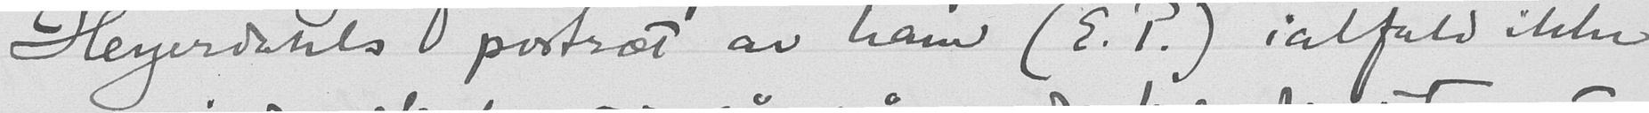Heyerdahls portræt av ham (E.P.) ialfald ikke
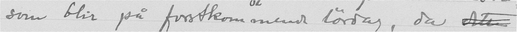som blir på forstkommende lørdag, da dette
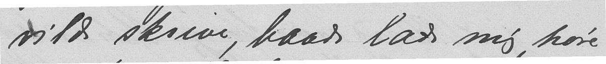vilde skrive, baade lade mig høre
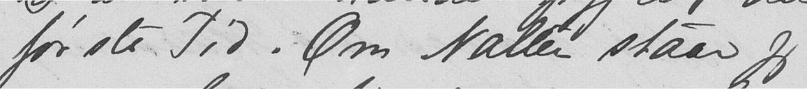første Tid. Om Natten staar jeg
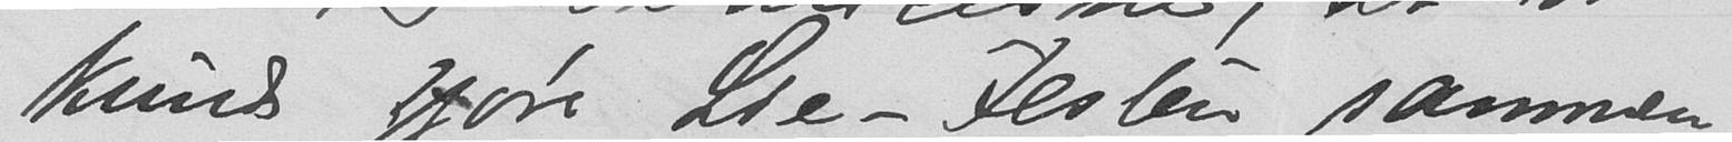kunde gjøre Lie-Festen sammen
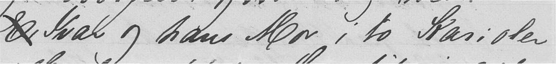E Ivar og hans Mor i to Karioler
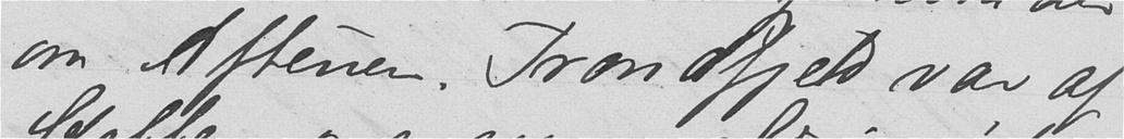om Aftenen. Trondfjeld var af
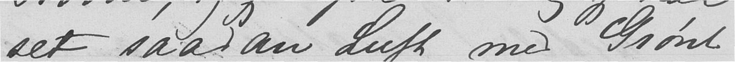det saadan Luft med Grønt
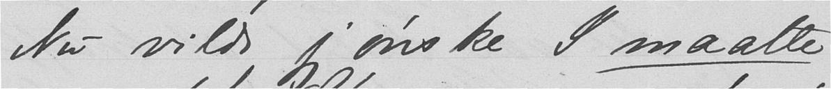Nu vilde jeg ønske I maatte
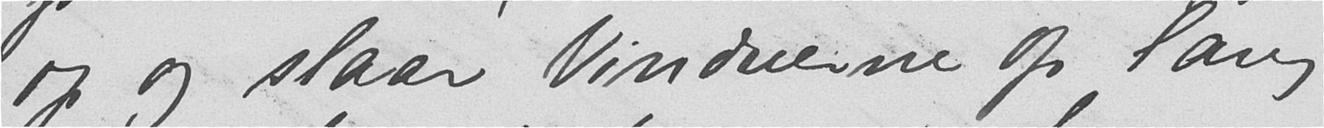op og slaar Vinduerne op lang
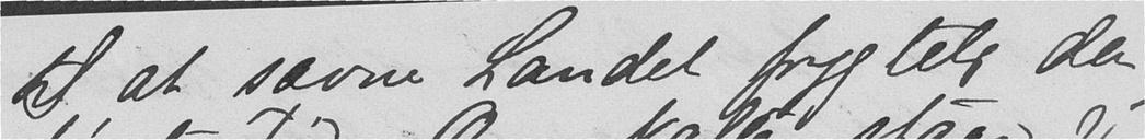til at savne Landet fygtelig den
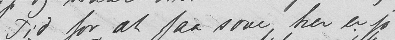Tid, for at faa sove, her er jo
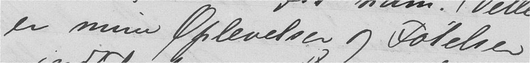er mine Oplevelser og Følelser
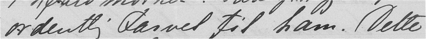ordentlig Farvel til ham. Dette
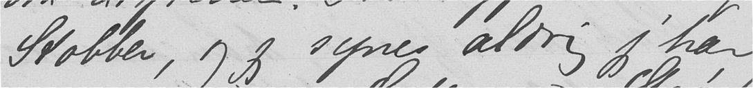Kobber, og jeg synes aldrig jeg har
